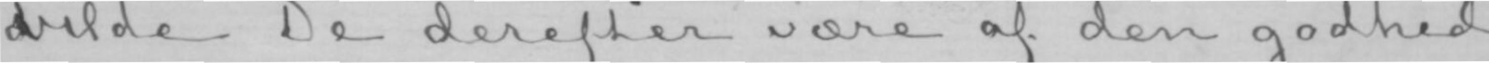dvilde De derefter være af den godhed
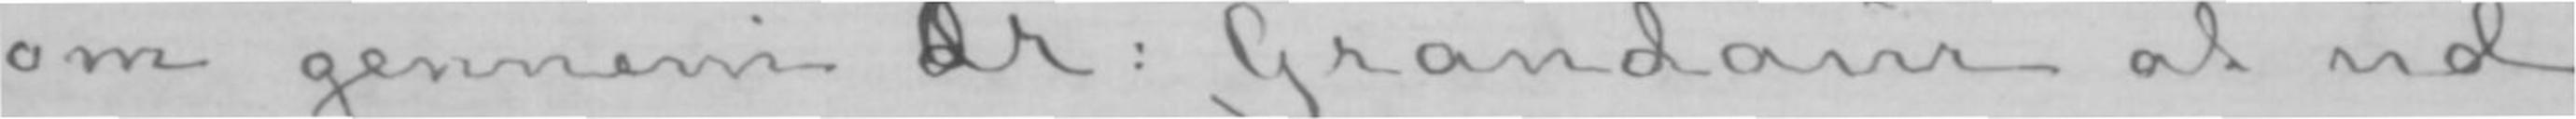om gennem dDr: Grandaur at ud-
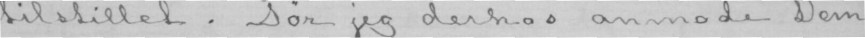tilstillet. Tør jeg derhos anmode Dem
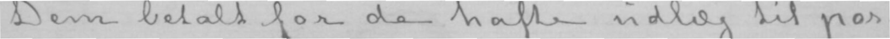Dem betalt for de hafte udlæg til por-
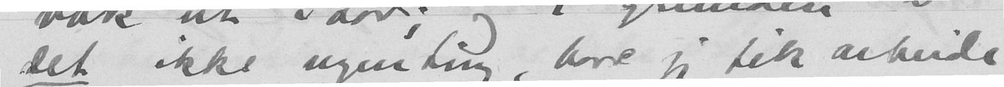det ikke nogenting, bare jeg fik arbeide
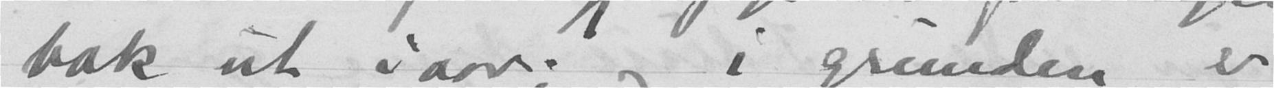bok ut iaar; i grunden er
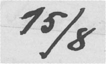15/8
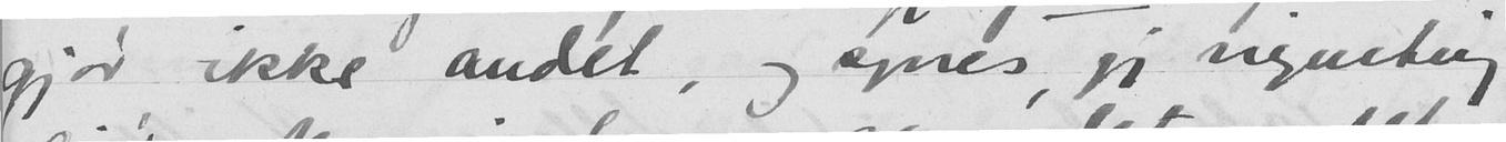gjør ikke andet, og synes jeg ingenting
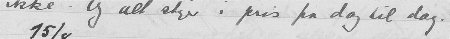ikke. Og alt stiger i pris fra dag til dag.
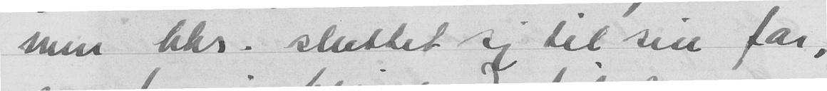men Chr. sluttet sig til sin far,
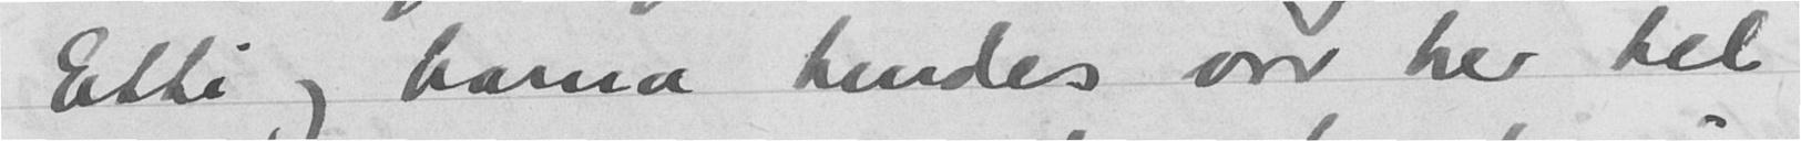Etti og barna hendes var her til
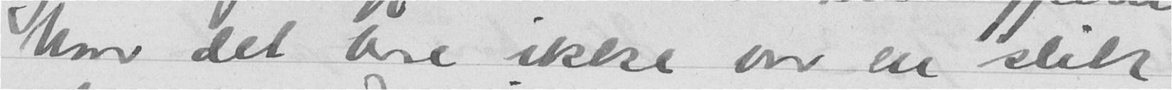Naar det bare ikke var en slik
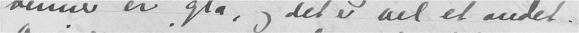venner er gla, og det er vel et andet.
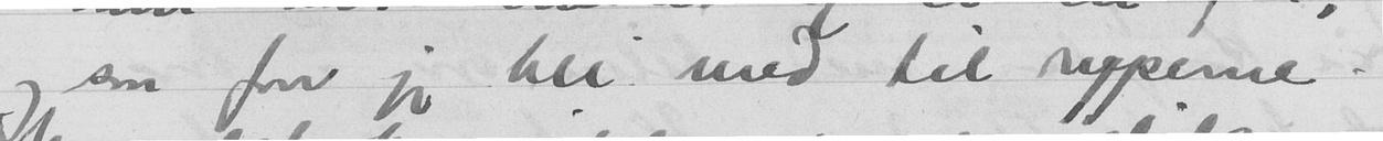og saa faar jeg bli med til ryperne.
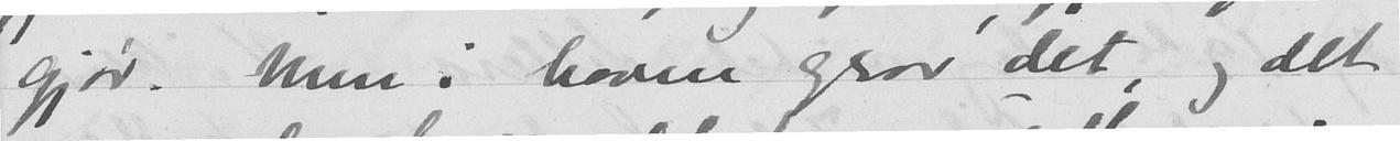gjør. Men i haven gror det, og det
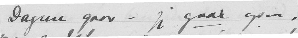Dagene gaar - jeg gaar ogsaa,
